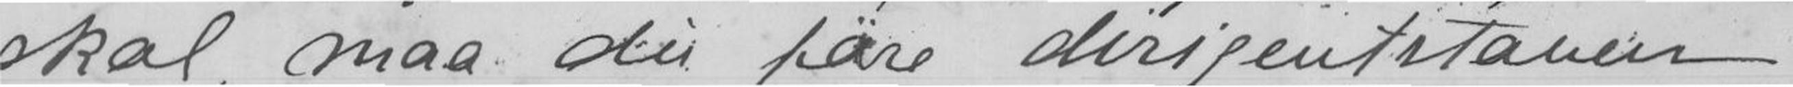skal, maa du føre dirigentstaven
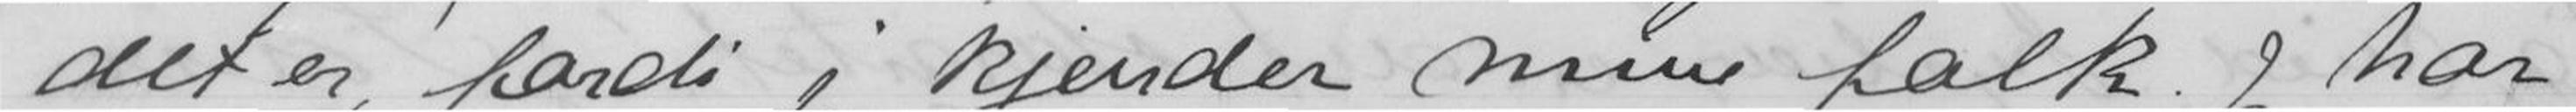det er fordi jeg kjender mine folk. Jeg har
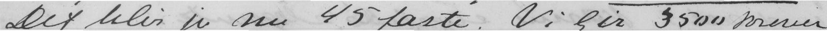Det blir jo nu 45 faste. Vi gir 3500 kroner
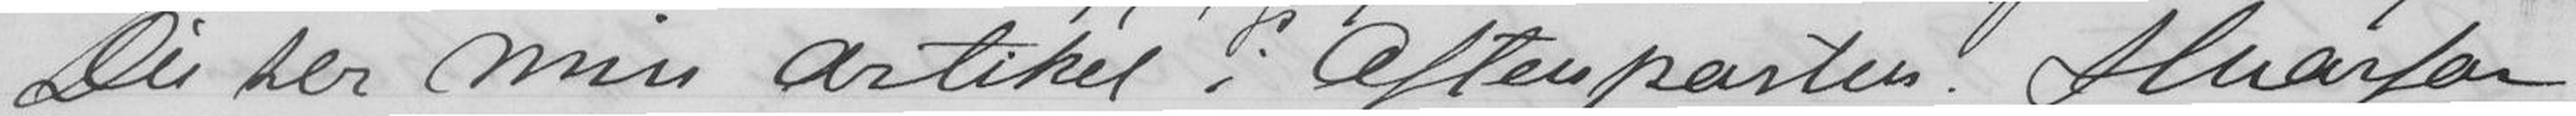Du ser min artikel i Aftenposten. Hvorfor
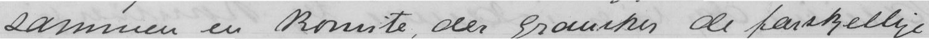sammen en komite, der gransker de forskillige
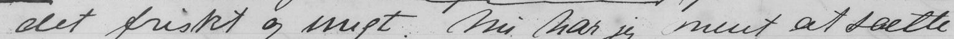det friskt og ungt. Nu har jeg ment at sætte
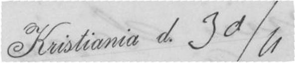Kristiania d. 30/11
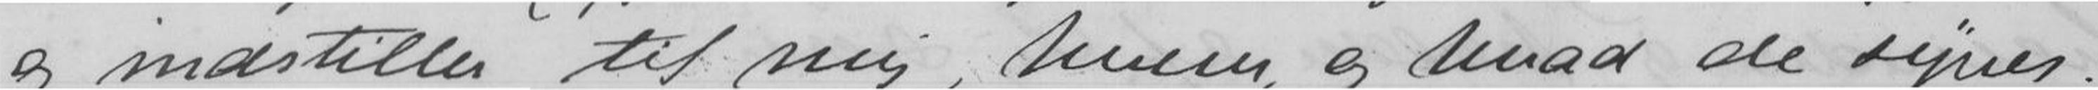og indstiller til mig, hvem, og hvad de synes.
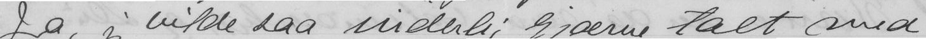Ja, jeg vilde saa inderlig gjærne
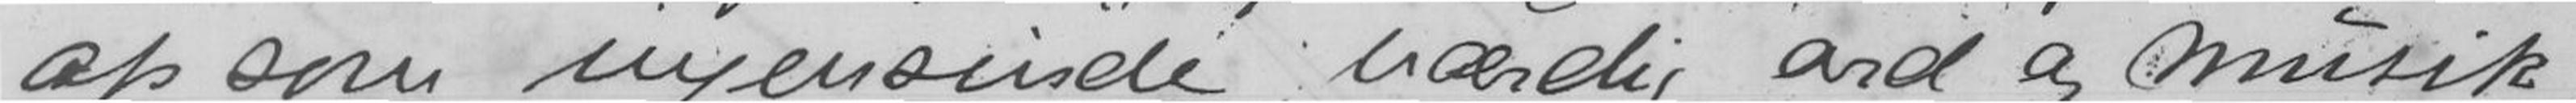op som ingensinde, værdig ord og musik;
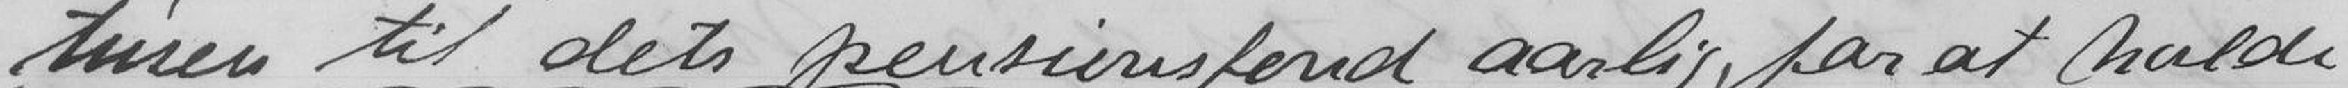tusentil dets pensionsfond aarlig, for at holde
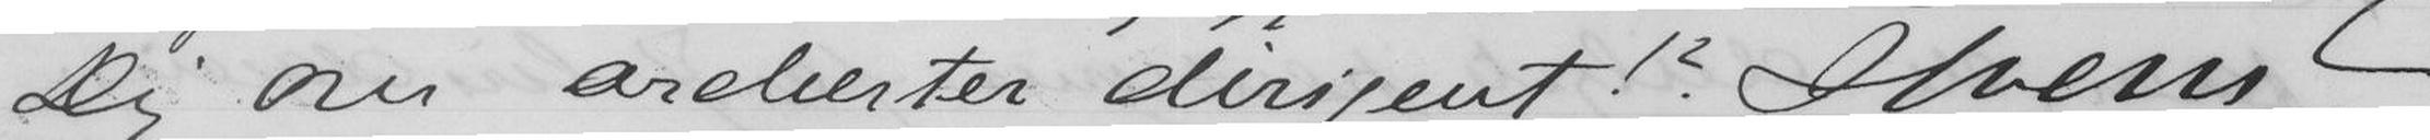talt med Dig om orkester dirigent!? Hvem?
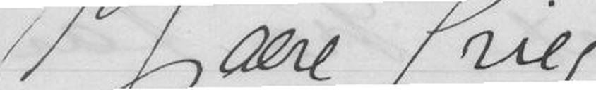Kjære Grieg
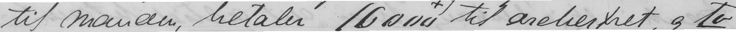til manden, betaler 16000x) til orchestret, og to
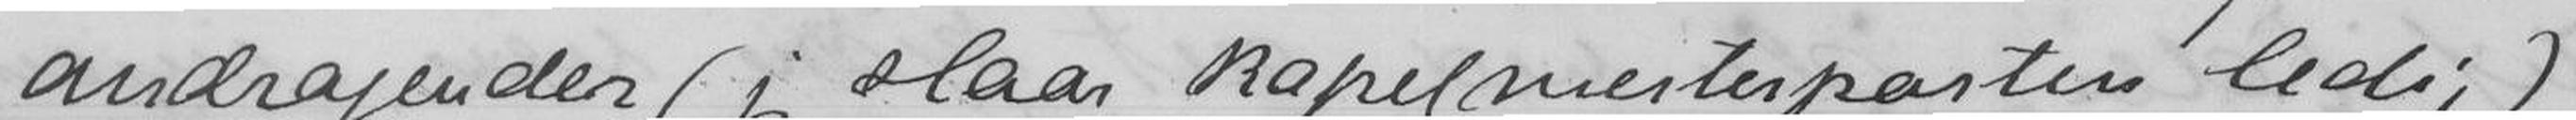andragender (jeg slaar kapelmesterposten ledig)
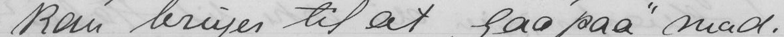kan bruges til at gaa paa mod!
